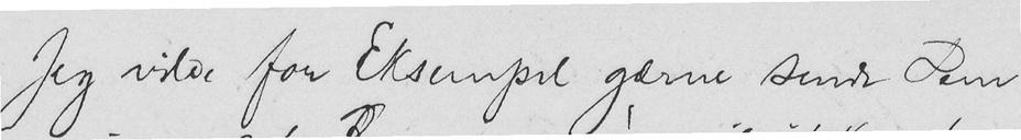Jeg vilde for Eksempel gærne sende Dem
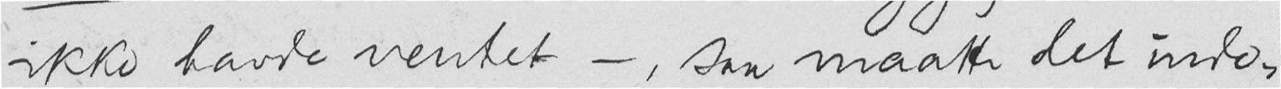ikke havde ventet -, saa maatte det inde-
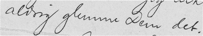aldrig glemme Dem det.
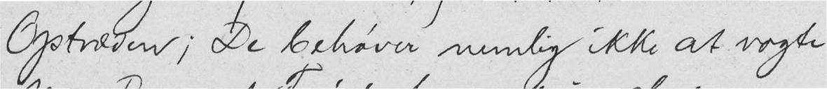Optræden; De behøver nemlig ikke at vogte
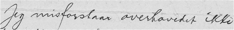Jeg misforstaar overhovedet ikke
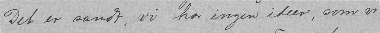Det er sandt, vi har ingen ideer, som vi
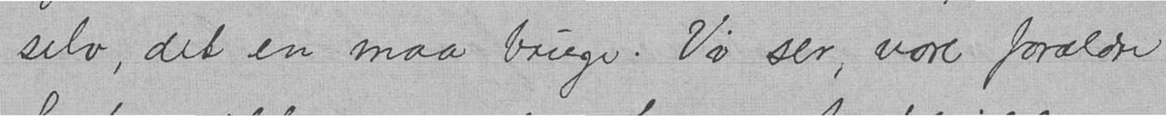selv, det en maa bruge. Vi ser, vore forældre
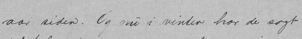aar siden. Og nu i vinter har de sagt
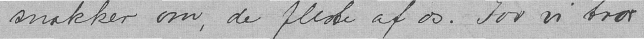snakker om, de fleste af os. For vi tror
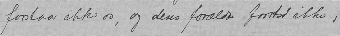forstaar ikke os, og deres forældre forstod ikke,
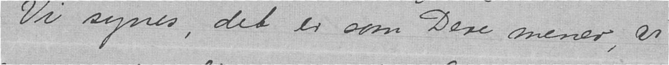Vi synes, det er som Dere mener vi
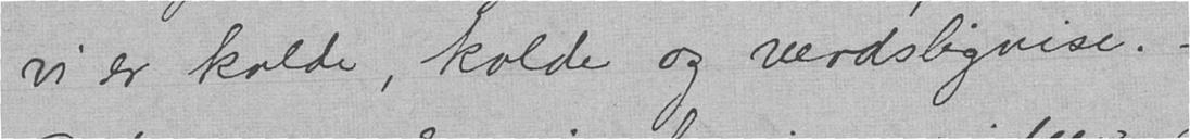vi er kolde, kolde og verdsligvise. -
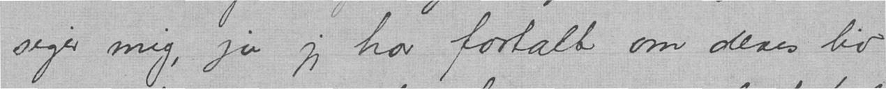siger mig, ja jeg har fortalt om deres liv
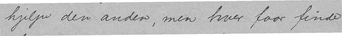hjelpe den anden, men hver faar finde
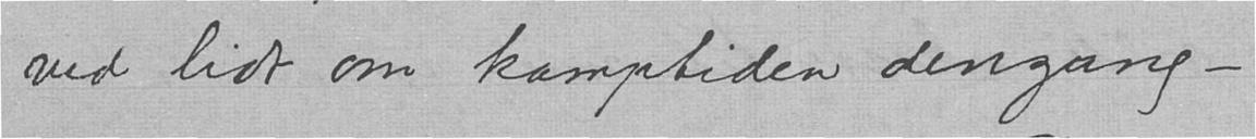ved lidt om kamptiden dengang -
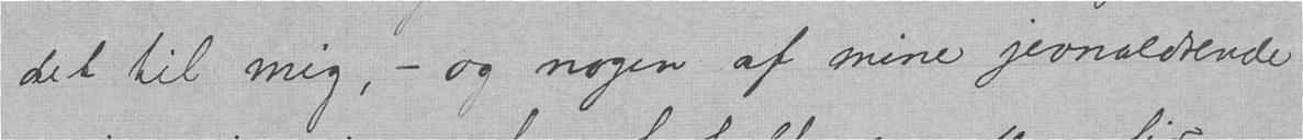det til mig, - og nogen af mine jevnaldrende
